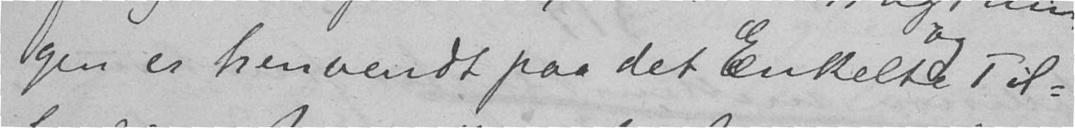gen er henvendt paa det Enkelte Til-
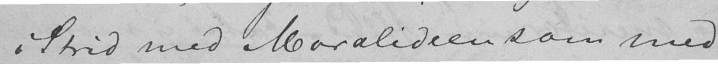i Strid med Moralideen som med
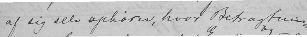af sig selv ophører, hvor Betragtnin-
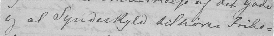og al Synderskyld tilhører Frihe-
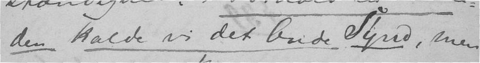den kalde vi det onde Synd, men
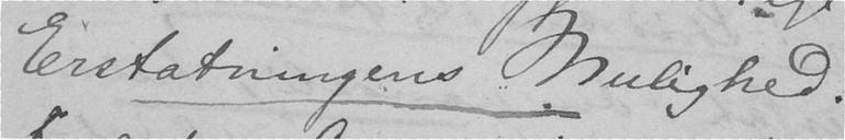Erstatningens Mulighed.
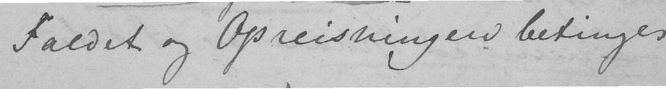Faldet og Opreisningen betinges
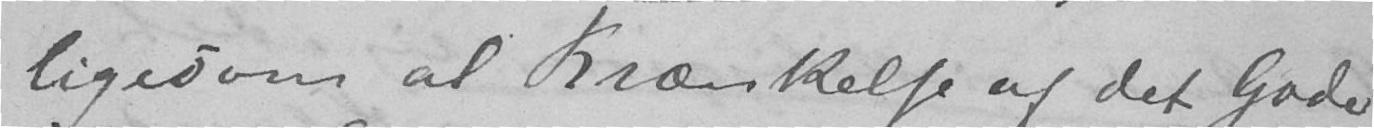ligesom al Krænkelse af det Gode
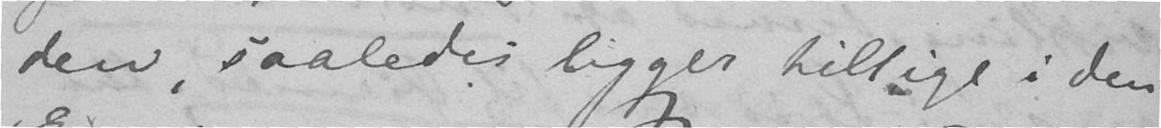den, saaledes ligger tillige i den
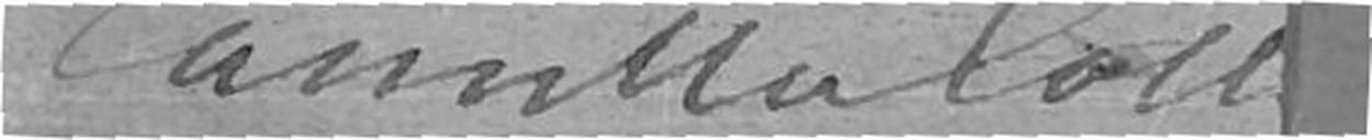Camilla Coll[ett]
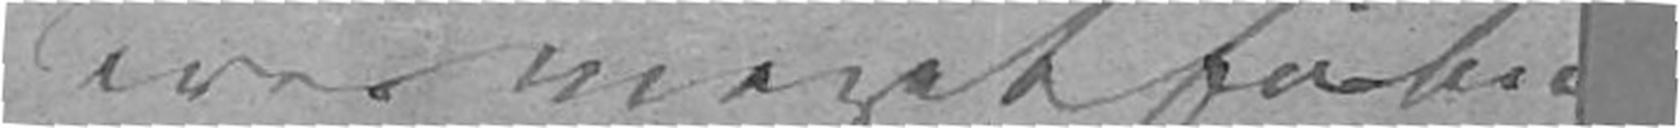Deres meget forbu[ndne]
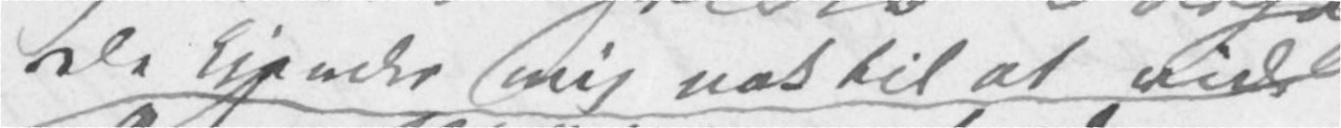De kjender mig nok til at vide
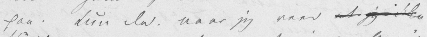paa. Kun da, naar jeg veed at jeg ikke
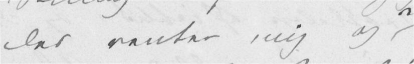der venter mig og
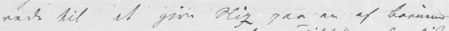rede til at give slip paa en af Børnene
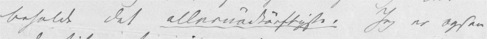beholde det allernødtørftigste. Jeg er ogsaa
